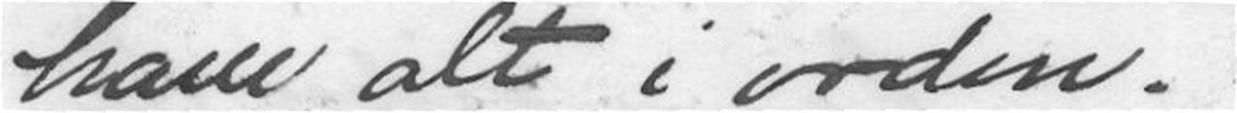have alt i orden.
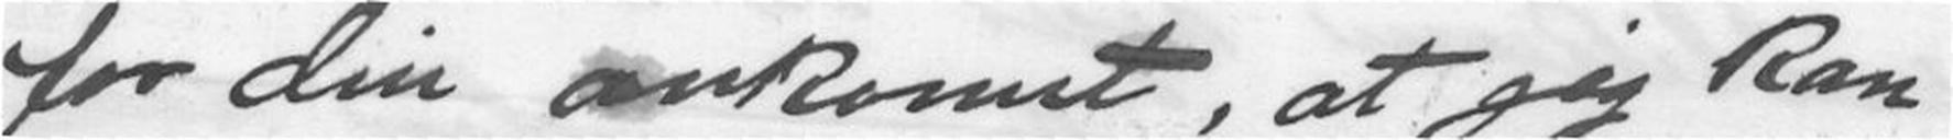for din ankomst, at jeg kan
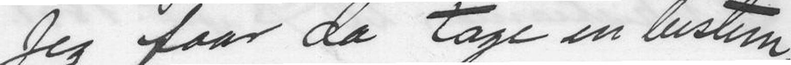Jeg faar da tage en bestem
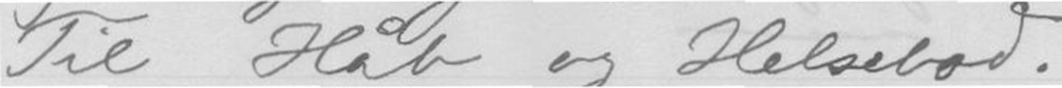Til Håb og Helsebod.
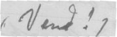(Vend!)
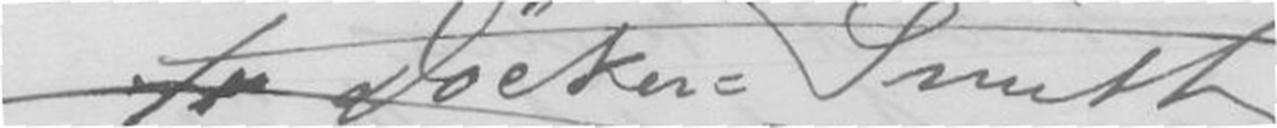Fr Döcker-Smith.
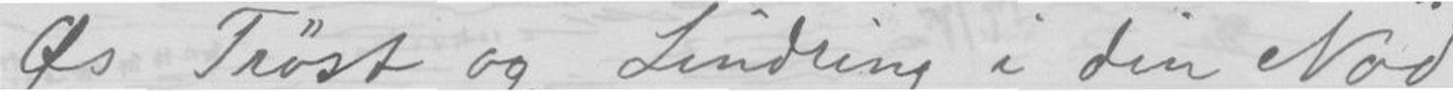Øs Trøst og Lindring i din Nød
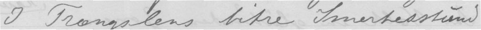I Trængslens bitre Smertesstund
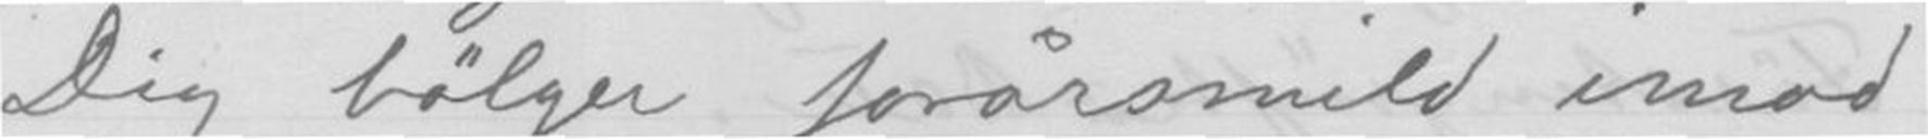Dig bølger forårsmild imod
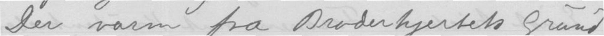Der varm fra Broderhjertets Grund
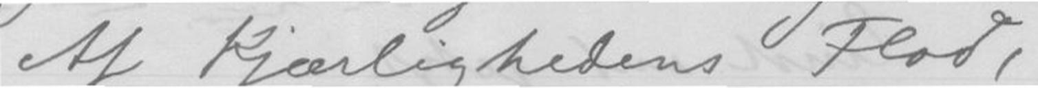Af Kjærlighedens Flod,
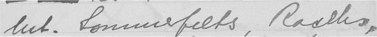lnt. Sommerfelts, Raschs,
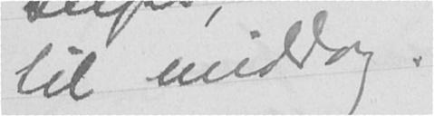til middag.
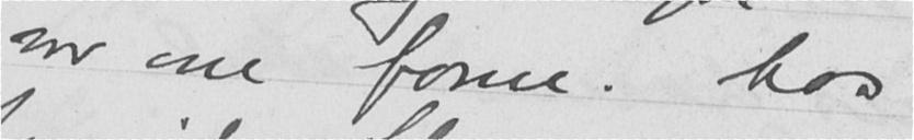var om form. hos
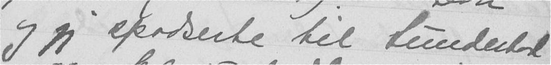og jeg spadserte til Lundestad
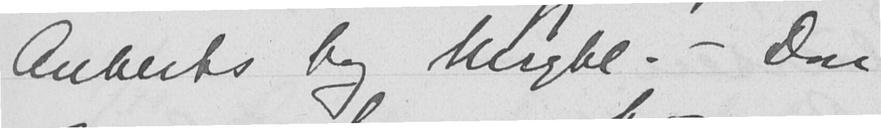Auberts bog Mrgbl. - Don
